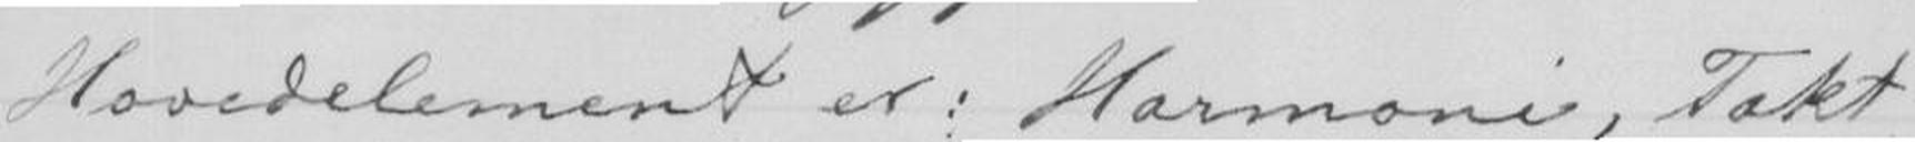Hovedelement er Harmoni, Takt
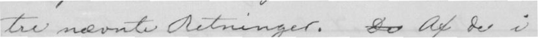tre nævnte Retninger. De Af de i
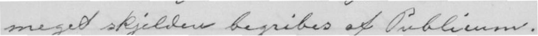meget skjelden begribes af Publikum.
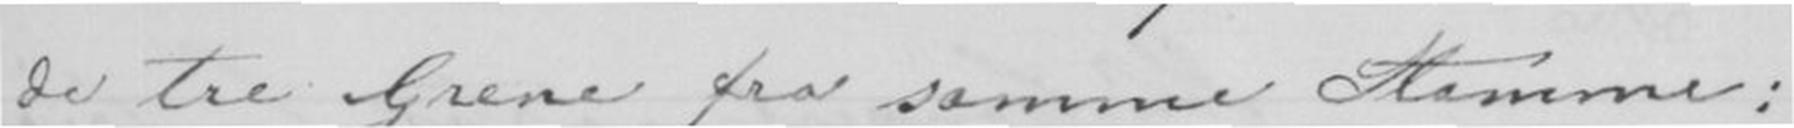de tre Grene fra samme stamme;
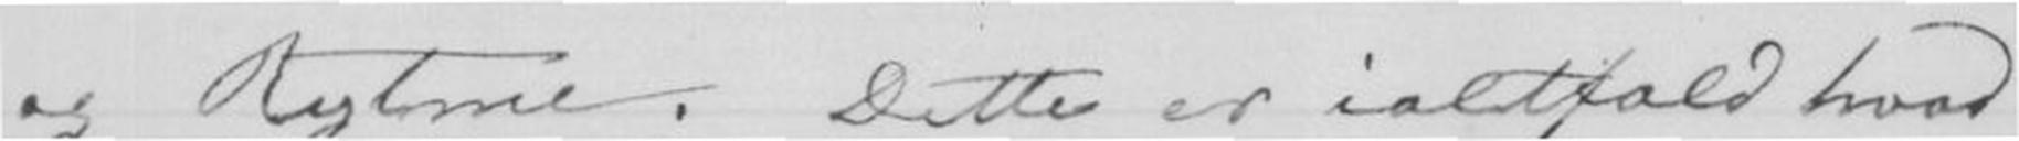og Rytme. Dette er ialtfald hvad
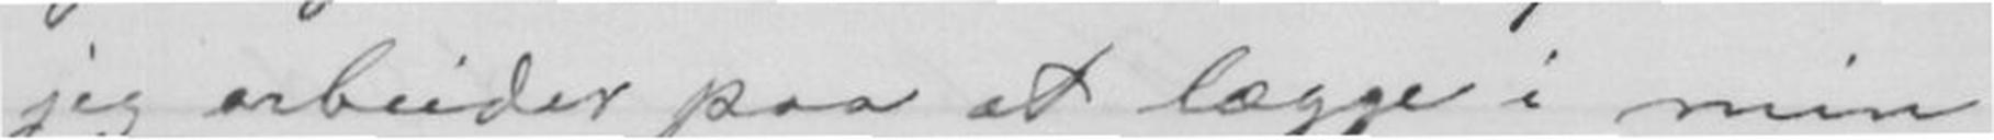jeg arbeider paa at lægge i min
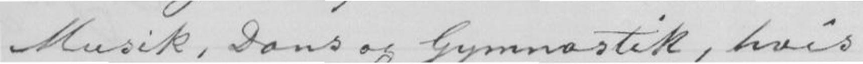Musik, Dans og Gymnastik, hvis
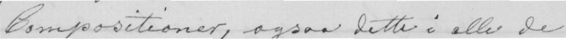Compositioner, ogsaa dette i alle de
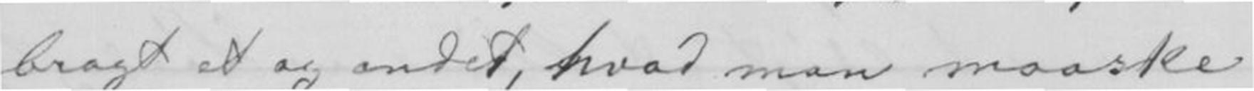bragt et og andet, hvad man maaske
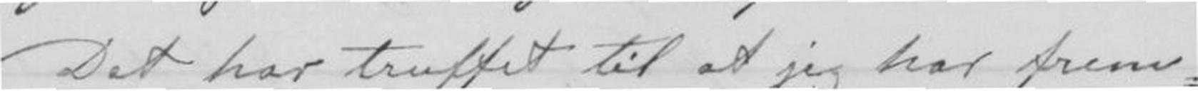Det har truffet til at jeg har frem-
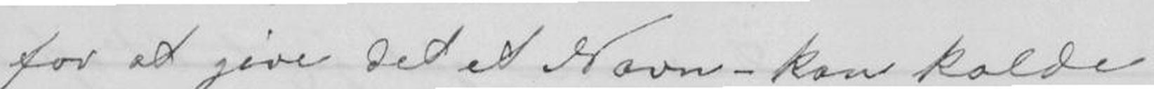for at give det et Navn- kan kalde
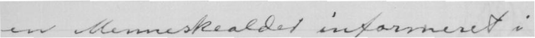en Menneskealder informeret i
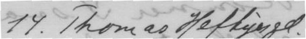14. Thomas Heftyesgd.
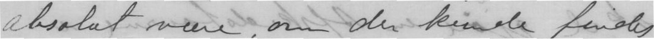absolut være, om der kunde findes
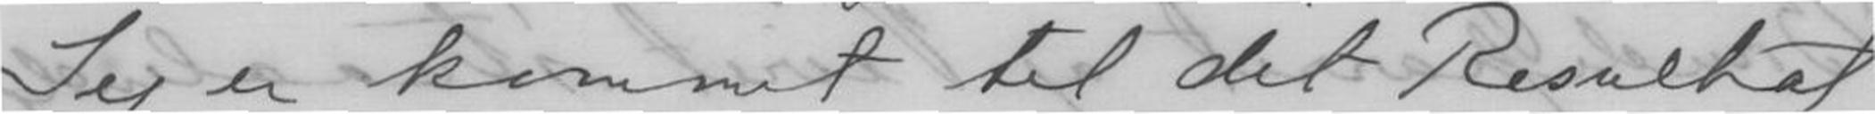Jeg er kommet til det Resultat,
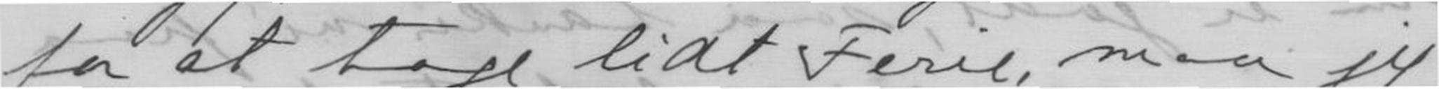for at tage lidt Ferie, maa jeg
